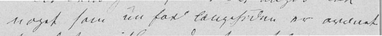noget som nu for længesiden er ordnet
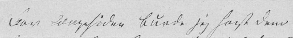For længesiden burde jeg sagt Dem
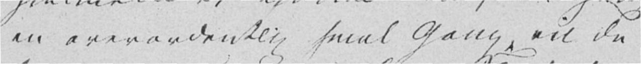en overordentlig smal Gang, vil De
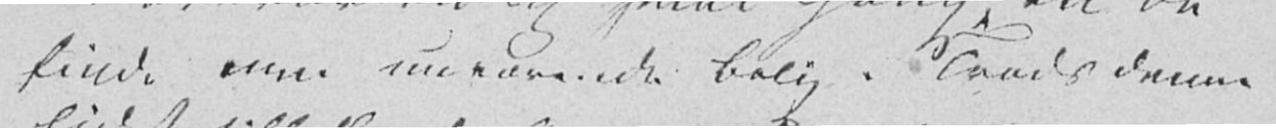finde min nuværende Bolig. Trods denne
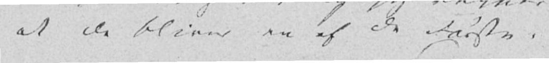at De bliver en af de Første.
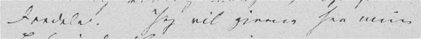Fordele. Jeg vil gjerne see mine
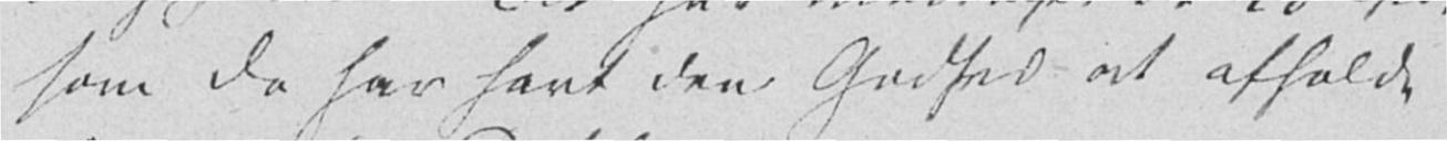som De har havt den Godhed at afholde
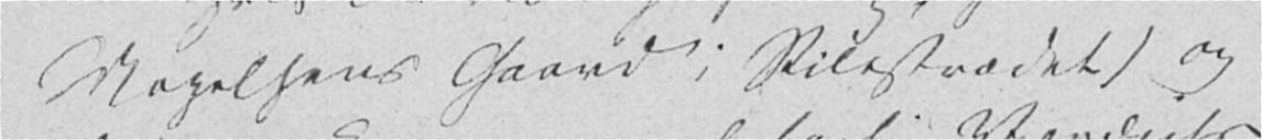Magelsens Gaard i Pilestrædet) og
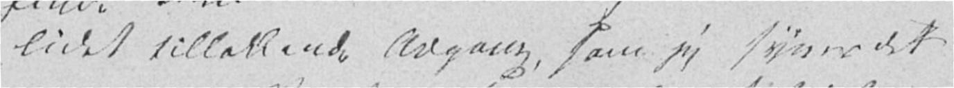lidet tillokkende Adgang, som jeg synes det
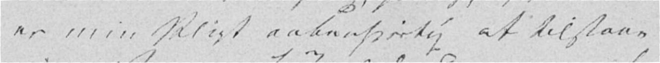er min Pligt aabenhjertig at tilstaae
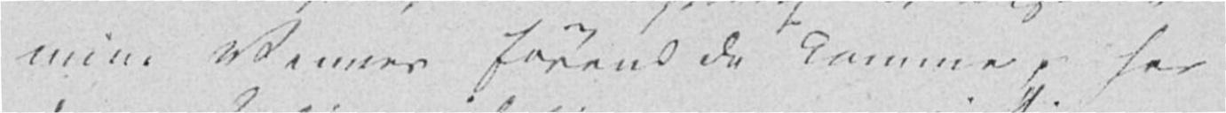mine Venner førend de komme, har
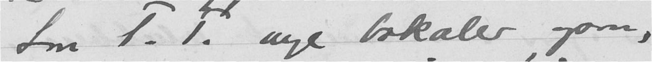Sm T. T. nye lokaler ogsaa,
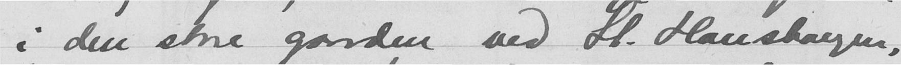i den store gaarden ved St. Hanshaugen,
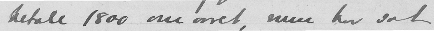betale 1800 om aaret, men har sat
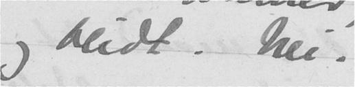og blidt. Nei.
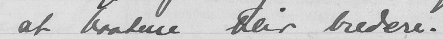at baatene blir bredere.
